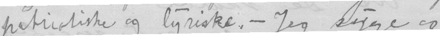patriotiske og lyriske. - Jeg syge og
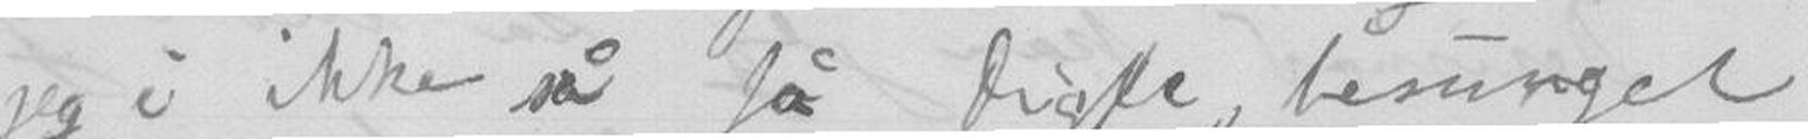jeg i ikke så få Digte, besunget
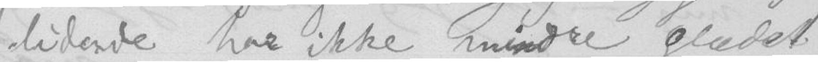lidende har ikke mindre glædet
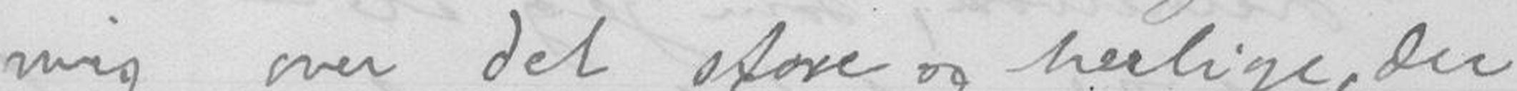mig over det store og herlige, der
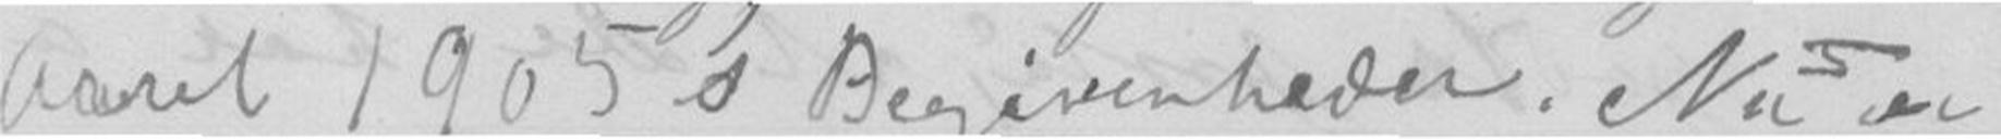Aaret 1905's Begivenheder. Nu er
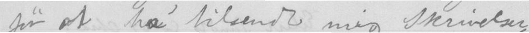før at há tilsendt mig skrivelser,
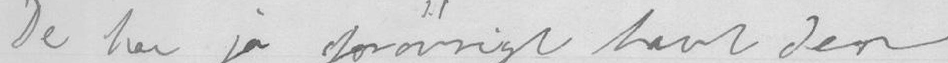De har jo forøvrigt havt den
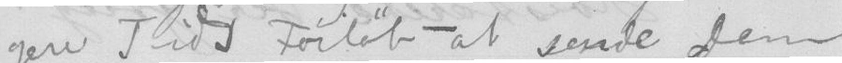gere Tids Førløb - at sende Dem
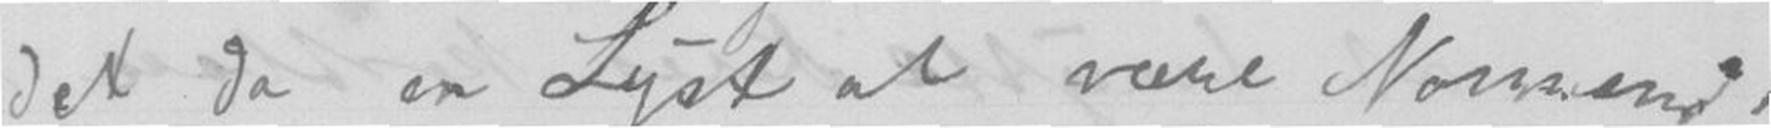Det da en Lyst at være Normand!
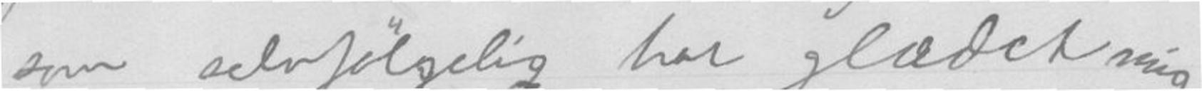som selvfølgelig har glædet mig
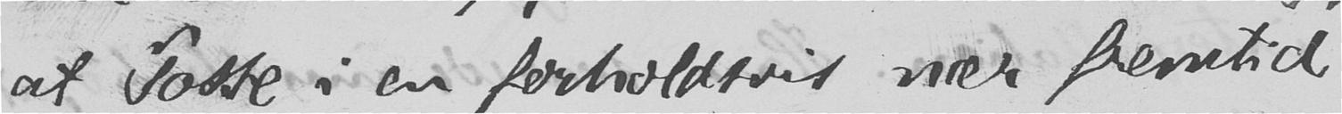at Fosse i en forholdsvis nær fremtid
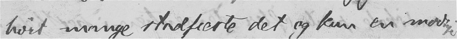hørt mange stadfæste det og kun en modsige
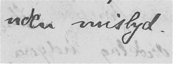uden mislyd.
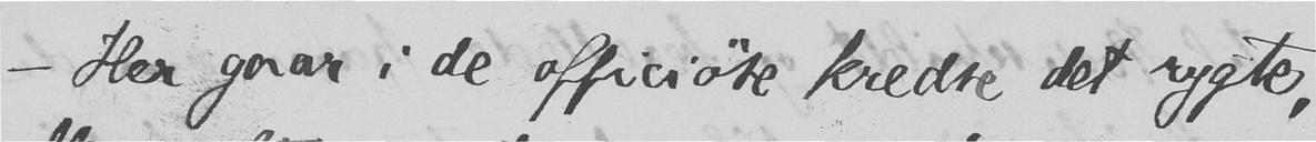– Her gaar i de officiøse kredse det rygte,
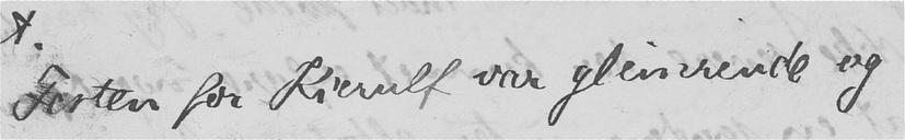Festen for Kierulf var glimrende og
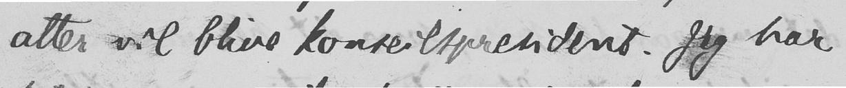atter vil blive konseilspresident. Jeg har
